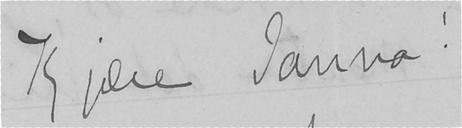Kjære Janna.
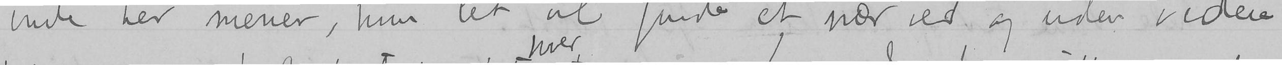inde her mener, hun let vil finde et nær ved og uden videre
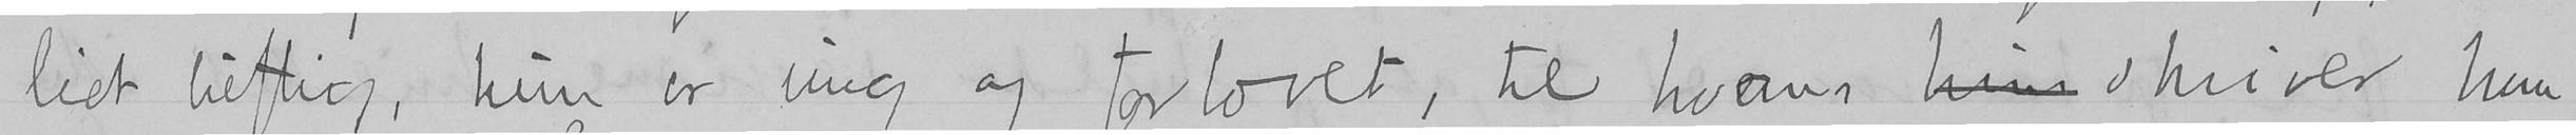lidt heftig, hun er ung og forlovet, til hvem hun skriver hun
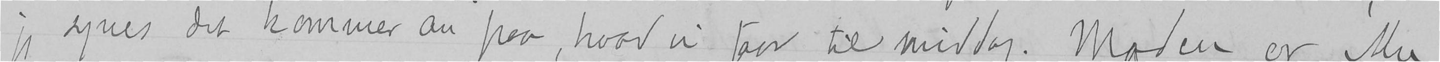jeg synes det kommer an paa, hvad vi faar til middag. Maden er ikke
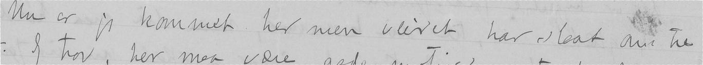Nu er jeg kommet her men veiret har slaat om til
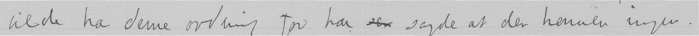vilde ha denne ordning for han ser sagde at der kommen ingen.
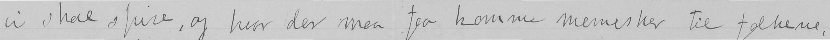vi skal spise, og hvor der maa faa komme mennesker til folkene,
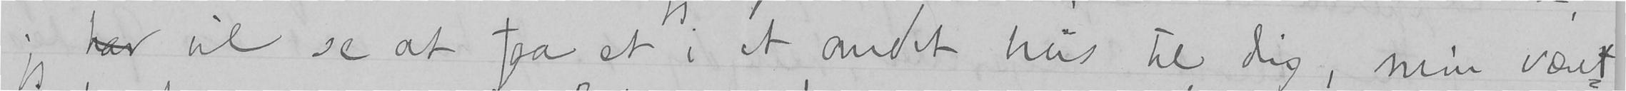jeg har vil se at faa et i et andet hus til dig, min vært-
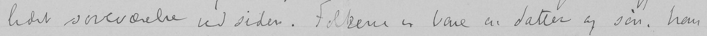lidet soveværelse ved siden. Folkene er bare en datter og søn, han
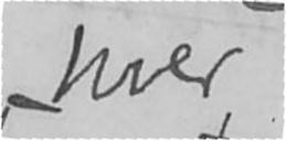hver
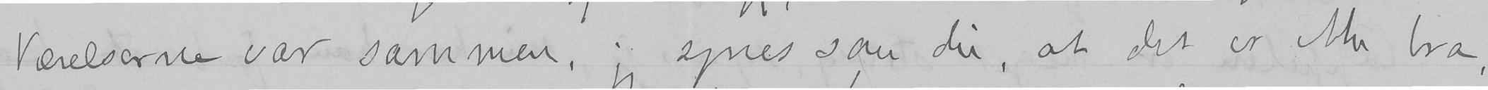Værelserne var sammen, jeg synes som du, at det er ikke bra,
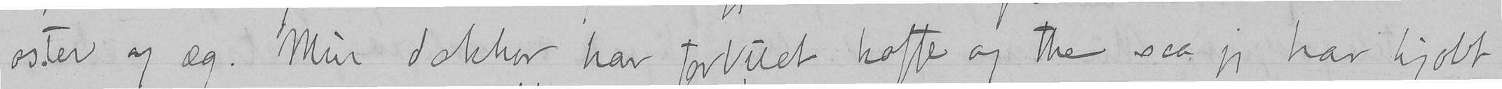oster og æg. Mun doktor har forbudt kaffe og the, saa jeg har kjøbt
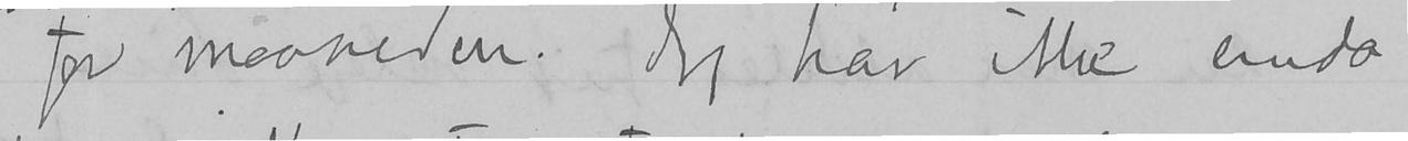for maaneden. Jeg har ikke enda
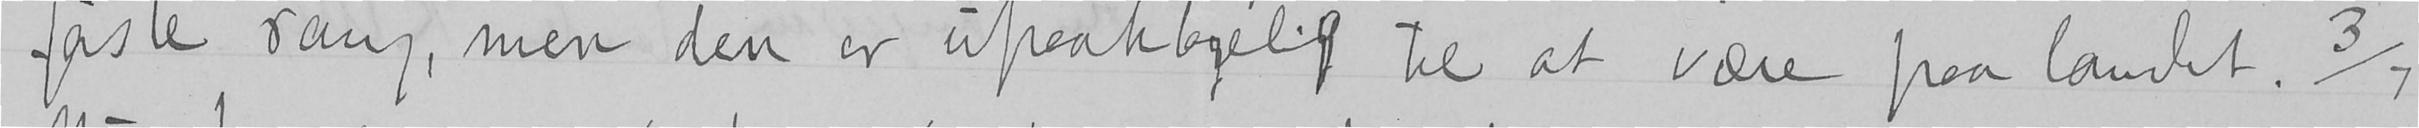første rang, men den er upaaklagelig til at være paa landet. 3/9
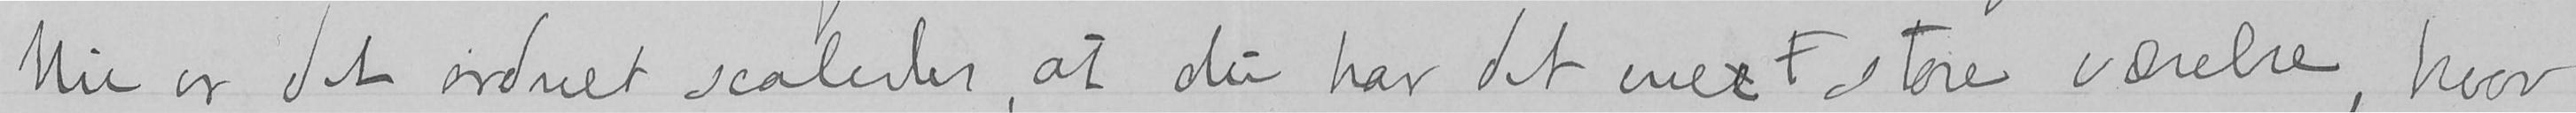Nu er det ordnet saaledes, at du har det enest store værelse, hvor
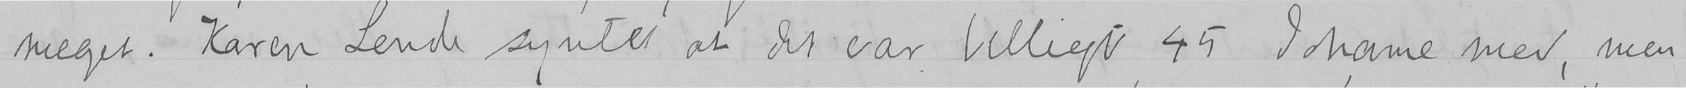meget. Karen Lende syntes at det var billigt 45 Johanne med, men
